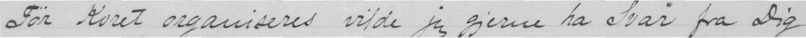Før Koret organiseres vilde jeg gjerne ha Svar fra Dig
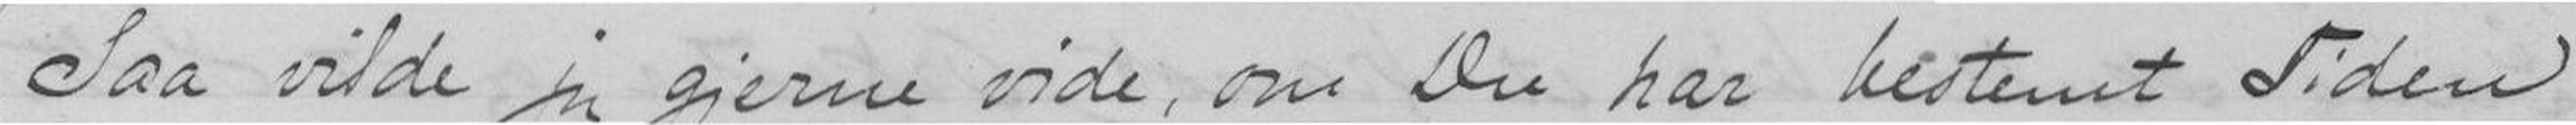Saa vilde jeg gjerne vide, om Du har bestemt Tiden
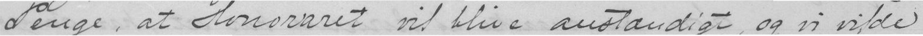Sange, at Honoraret vil blive anstændigt, og vi vilde
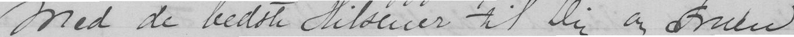Med de bedste Hilsener til Dig og Fruen
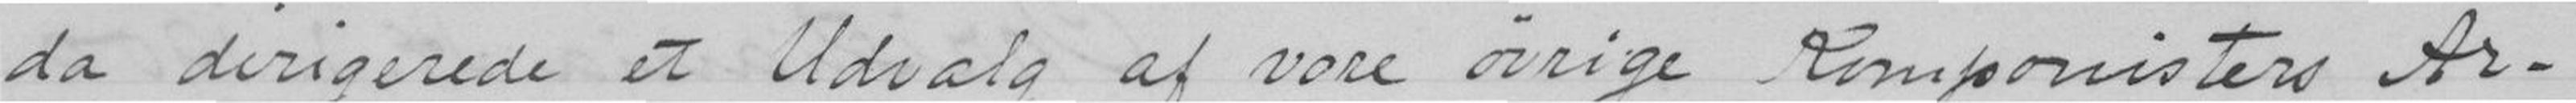da dirigerede et Udvalg af vore øvrige Komponisters Ar-
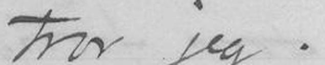Tror jeg.
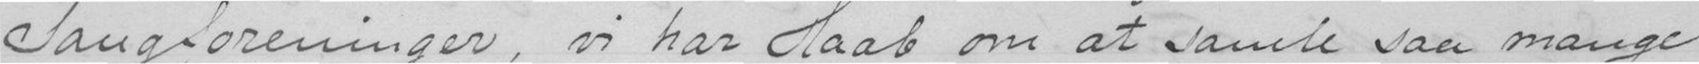Sangforeninger, vi har Haab om at samele saa mange
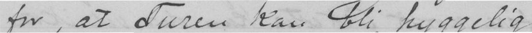for, at Turen kan bli hyggelig.
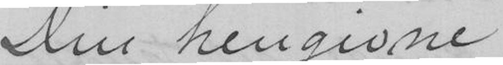Din hengivne
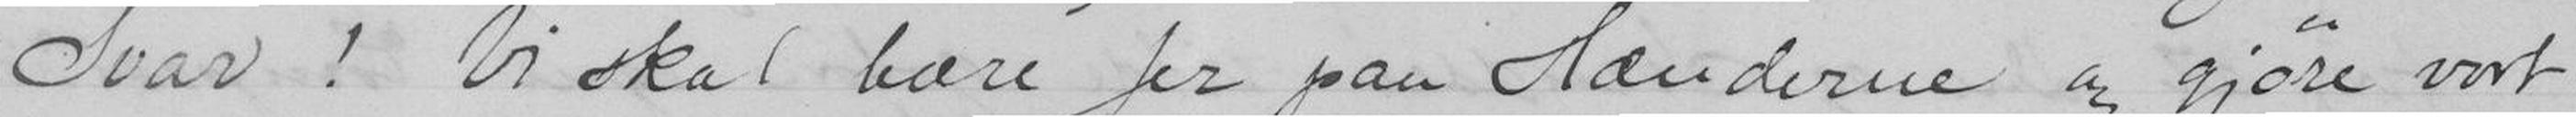Svar! Vi skal lære for paa Hænderne og gjøre vort
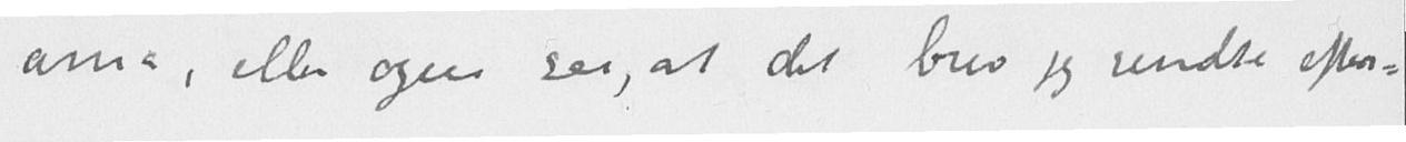ania, eller ogsaa saa, at det brev jeg sendte efter-
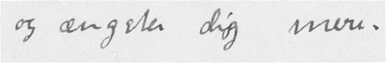og ængster dig mere.
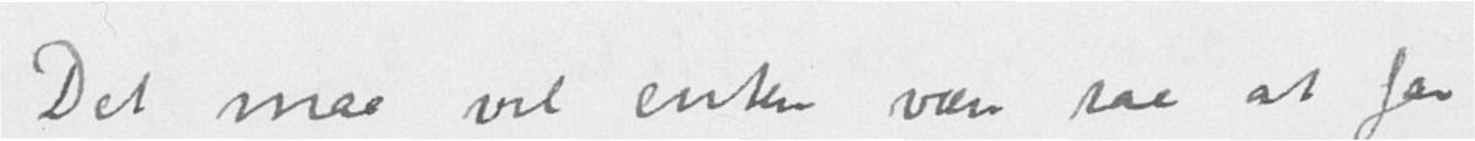Det maa vel enten være saa at far
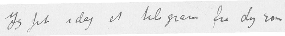Jeg fik i dag et telegram fra dig som
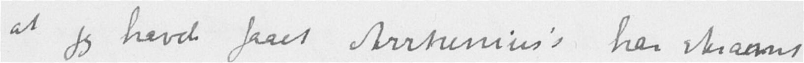at jeg havde faaet Arrhenius's har skræmt
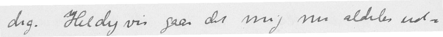dig. Heldigvis gaar det mig nu aldeles ud-
